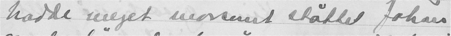hadde meget morsomt støttet Johan
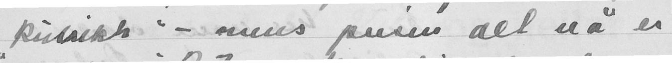kulsikk - mens prisen alt nå er
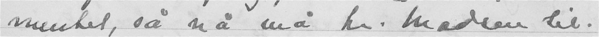mentet, så nå må hr. Madsen til.
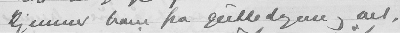kjenner ham fra guttedagene og vet,
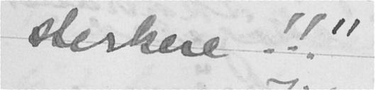sikrere!!."
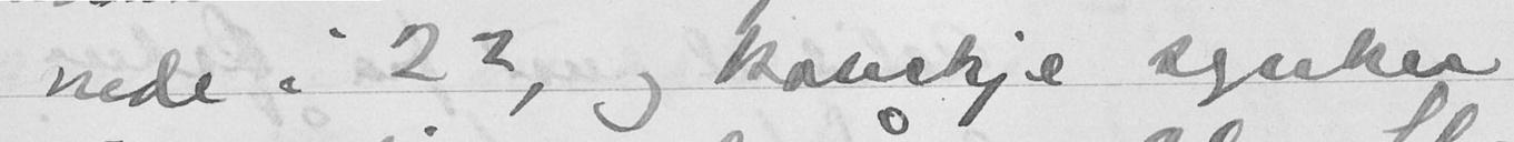nede i 22, og kanskje synker
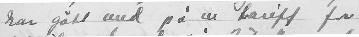har gått med på en tariff for
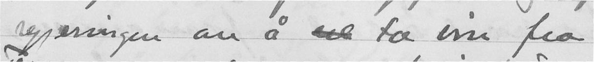regjeringen om å  ta vin fra
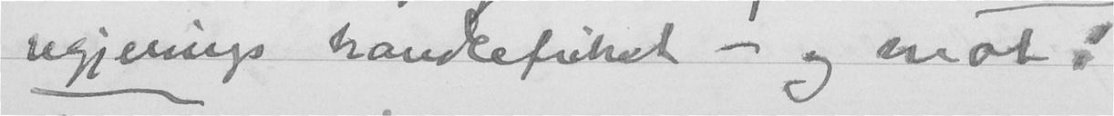regjerings handlefrihet - og mot!
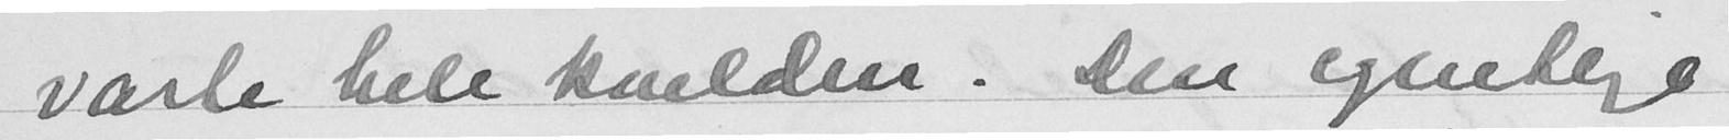varte hele kvelden. Den egentlige
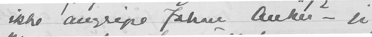ikke angripe Johan Anker - jeg
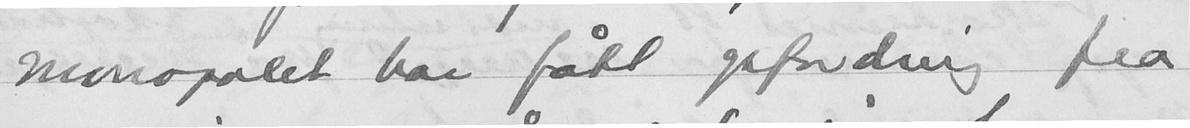monopolet har fått opfording fra
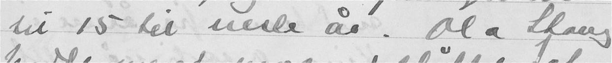til 15 til neste år. Ola Stang
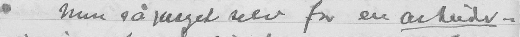Men såmeget selv for en arbeider-
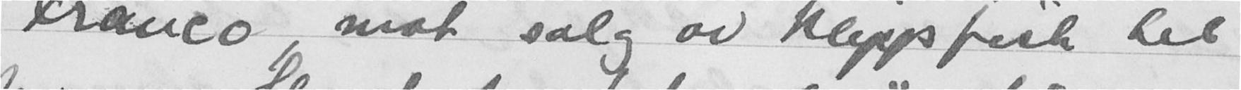Franco mot salg av klippfisk til
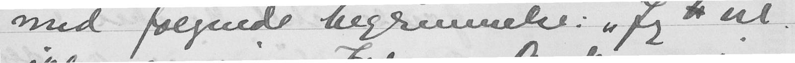med følgende begrunnelse. "Jeg  vil
